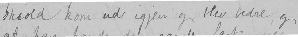skiold kom ud igjen og blev bedre, og
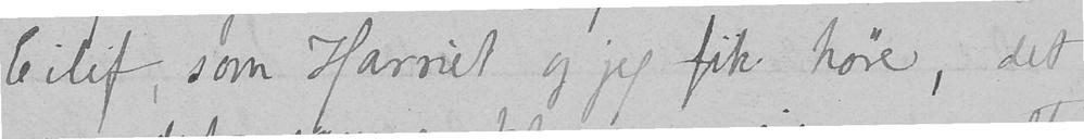Eilif, som Harriet og jeg fik høre, det
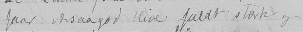faar værsaagod blive fuldt stærk og
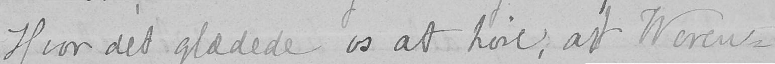Hvor det glædede os at høre, at Weren-
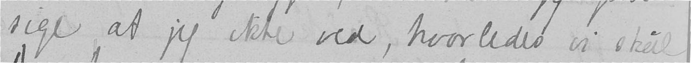sige, at jeg ikke ved, hvorledes vi skul-
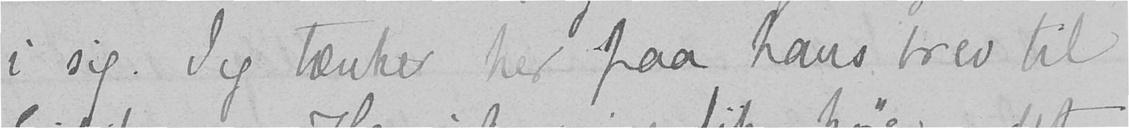i sig. Jeg tænker her paa hans brev til
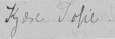Kjære Sofie!
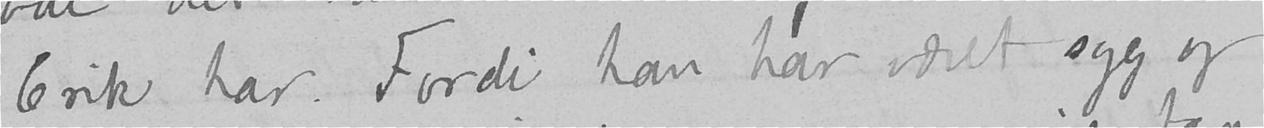Erik har. Fordi han har været syg og
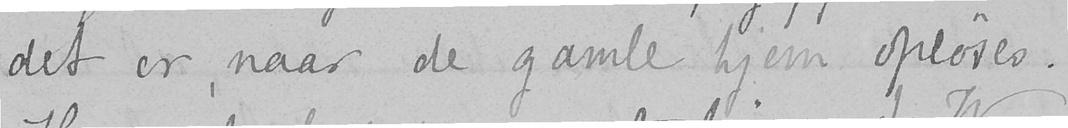det er, naar de gamle hjem opløses.
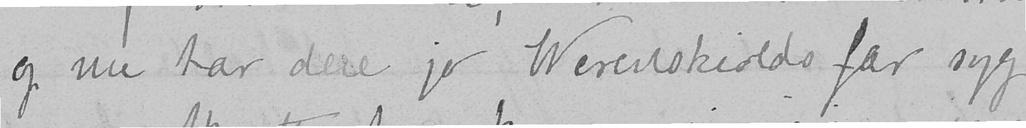og nu har dere jo Werenskiolds far syg
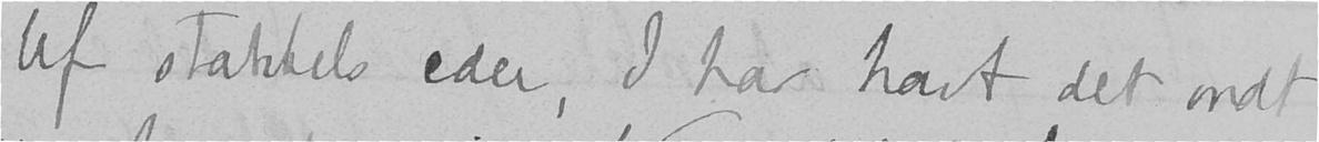Uf stakkels eder, I har havt det ondt
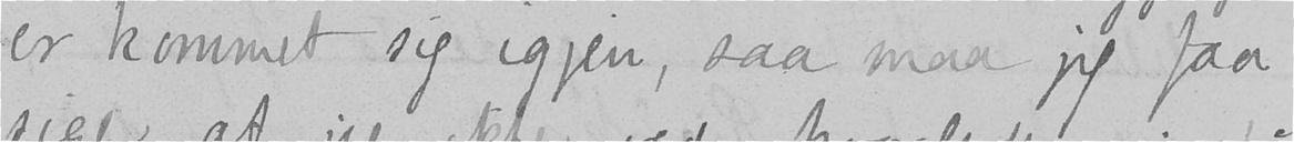er kommet sig igjen, saa maa jeg faa
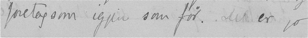foretagsom igjen som før. Det er jo
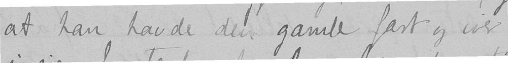at han havde den gamle fart og iver
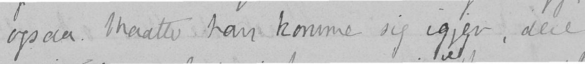ogsaa. Maatte han komme sig igjen, dere
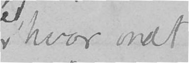hvor ondt
